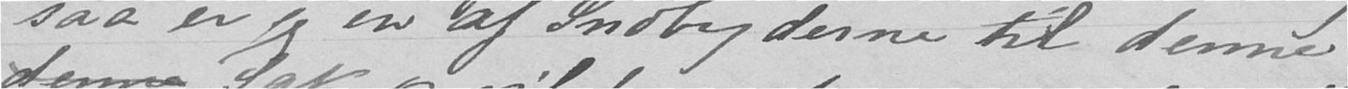saa er jeg en af Indbyderne til denne
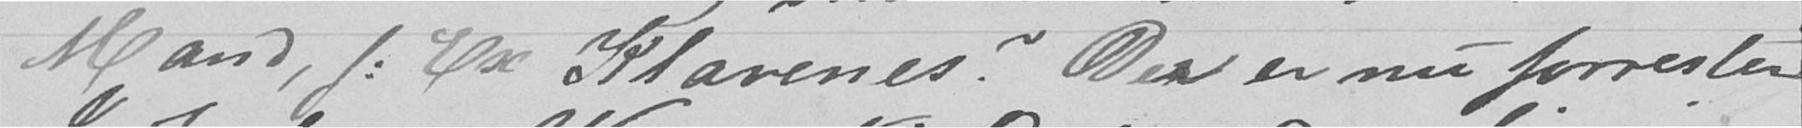Mand, f: Ex Klavenes? Der er nu forresten
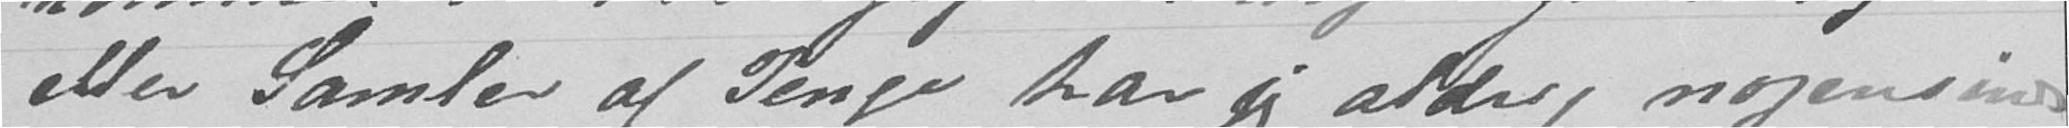eller Samler af Penge har jeg aldrig nogensinde
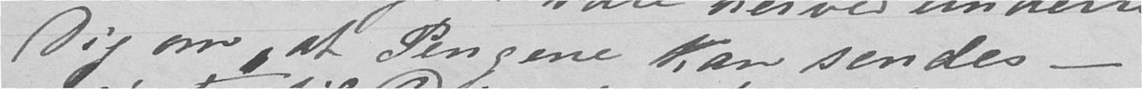Dig om, at Pengene kan sendes -
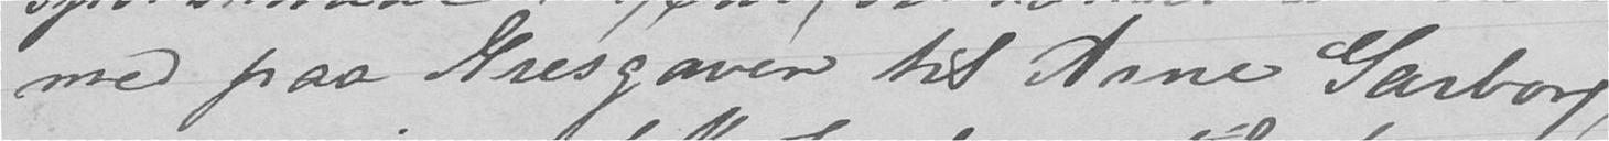med paa Æresgaven til Arne Garborg,
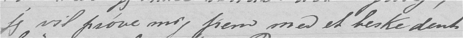jeg vil prøve mig frem med et beskedent,
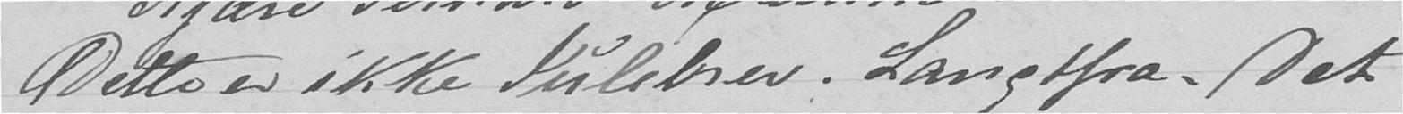Dette er ikke Julebrev. Langtfra. Det
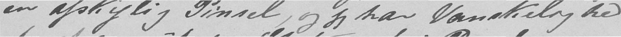en afskylig Pinsel, og jeg har Vanskelighed
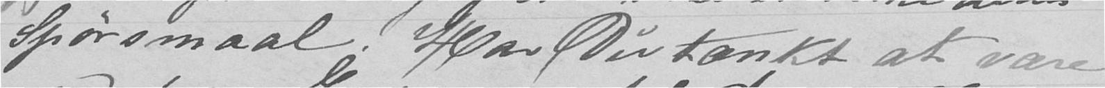Spørsmaal. Har Du tænkt at være
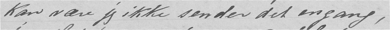kan være jeg ikke sender det engang,
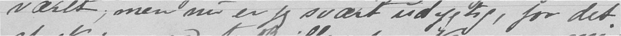været, men nu er jeg svært udygtig, for det
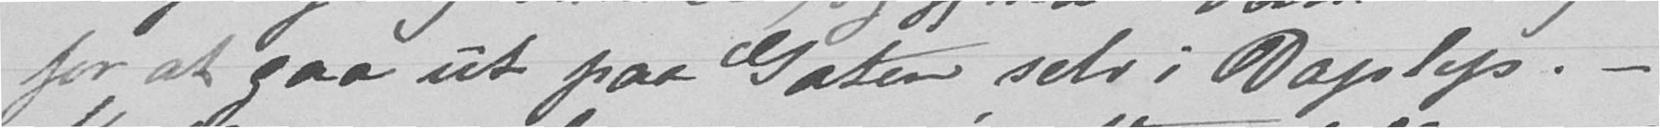for at saa ut paa Gaten selv i Dagslys.-
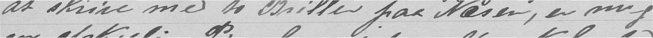at skrive med to Briller paa Næsen, er mig
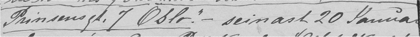Prinsensgt. 7 Oslo" - seinast 20 Januar
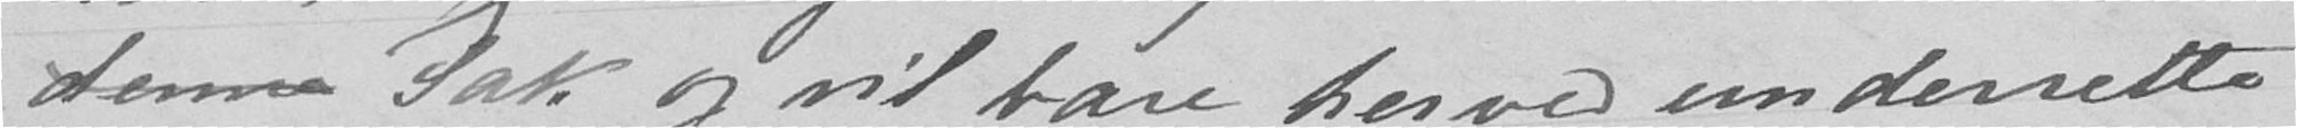denne Sak og vil bare herved underrette
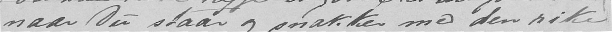naar Du staar og snakker med den rike
